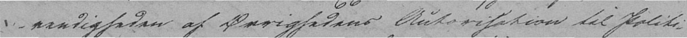vendigheden af Øvrighedens Autorisation til Politi-
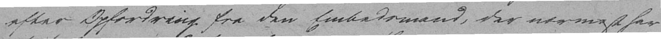efter Opfordring fra den Embedsmand, der nærmest har
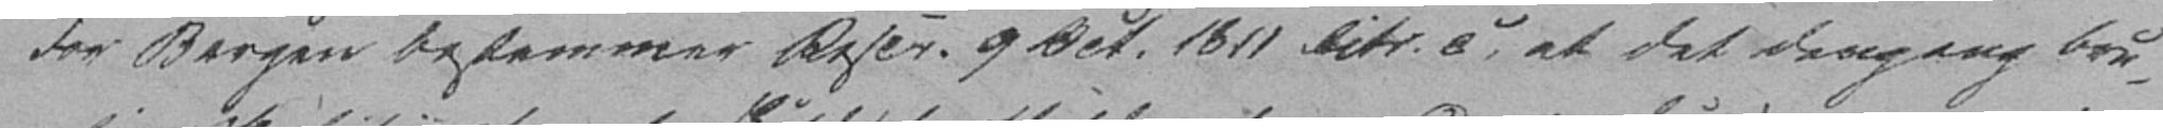For Bergen bestemmer Rescr. 9 Oct. 1811 litr. c, at det dengang bru-
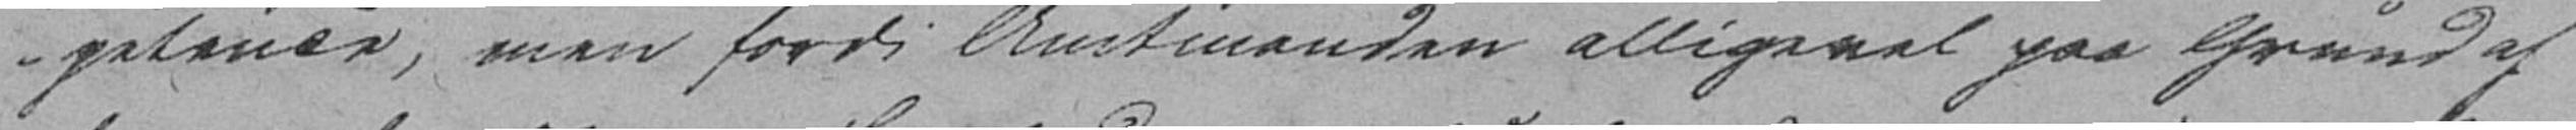petence, men fordi Amtmanden alligevel paa Grund af
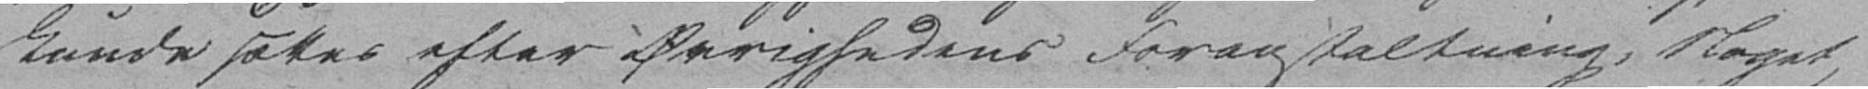kunde sættes efter Øvrighedens Foranstaltning, Noget,
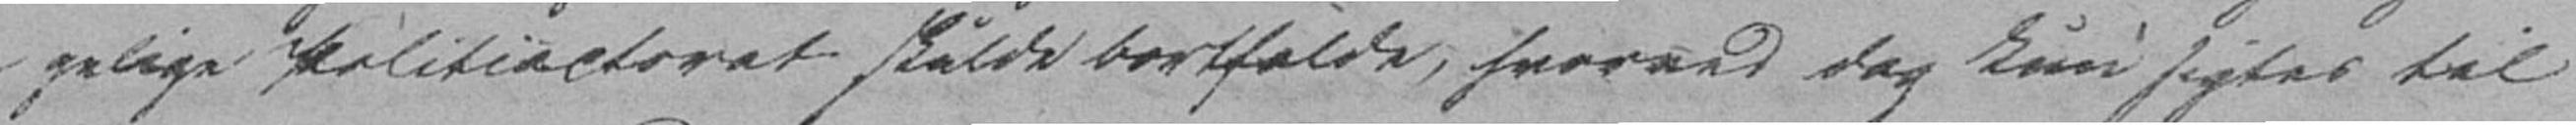gelige Politiactorat skulde bortfalde, hvorved dog kun sigtes til
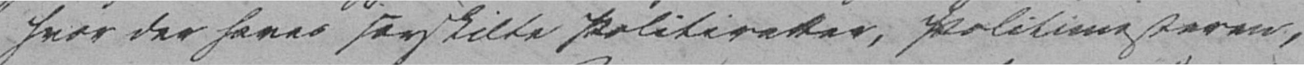hvor der haves særskilte Politiretter, Politimesteren,
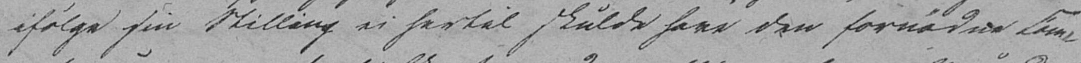ifølge sin Stilling ei hertil skulde have den fornødne Com-
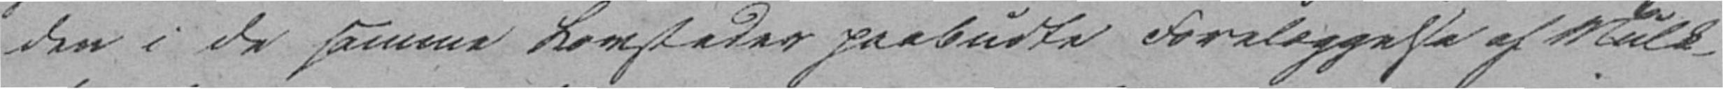den i de samme Lovsteder paabudte Forelæggelse af Mulk-
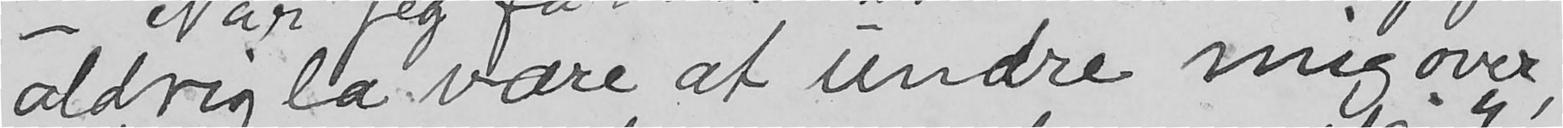aldrig la være at undre mig over,
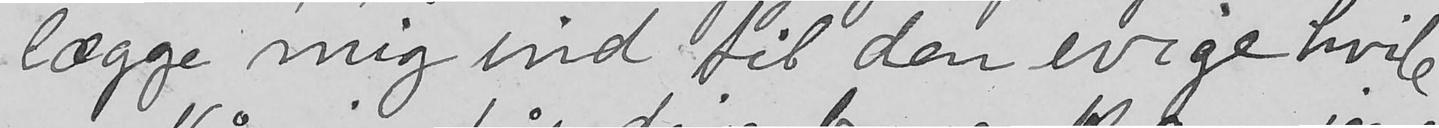lægge mig ind til den evige hvile.
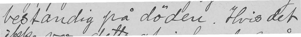bestandig på døden. Hvis det
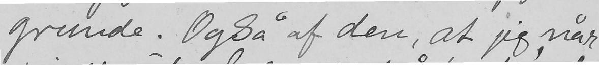grunde. Også af den, at jeg, når
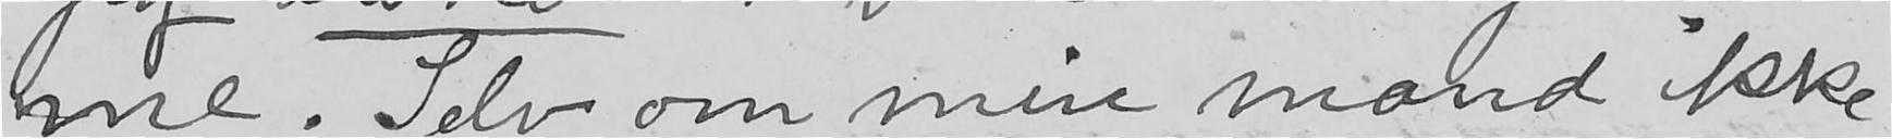me. Selv om min mand ikke
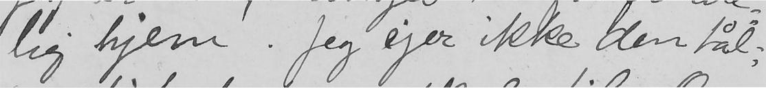lig hjem. Jeg ejer ikke den tål-
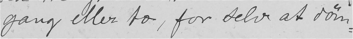gang eller to, for selv at døm-
